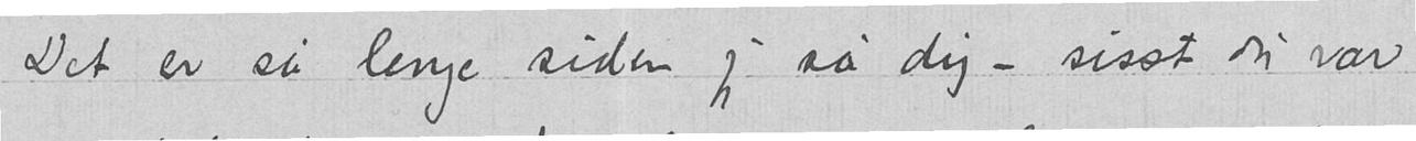Det er så lenge siden jeg sa dig – sisst du var
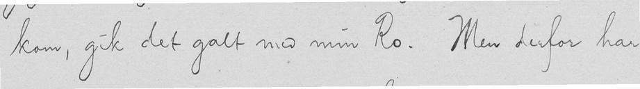kom, gik det galt med min Ro. Men derfor har
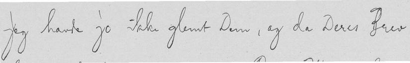jeg havde jo ikke glemt Dem, og da Deres Brev
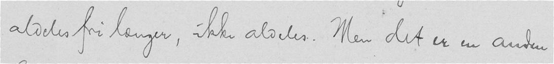aldeles fri længer, ikke aldeles. Men det er en anden
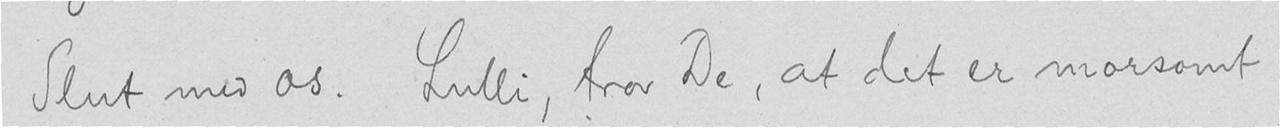Slut med os. Lulli, tror De, at det er morsomt
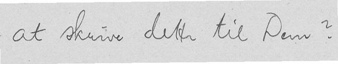at skrive dette til Dem?
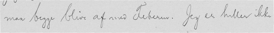maa begge blive af med Feberen. Jeg er heller ikke
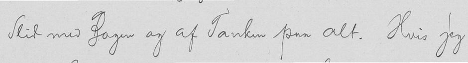Slid med Bogen og af Tanken paa alt. Hvis jeg
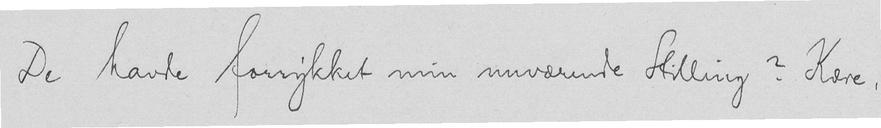De havde forrykket min nuværende Stilling? Kære,
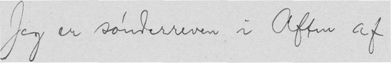Jeg er sønderreven i Aften af
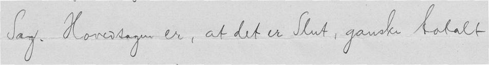Sag. Hovedsagen er, at det er Slut, ganske totalt
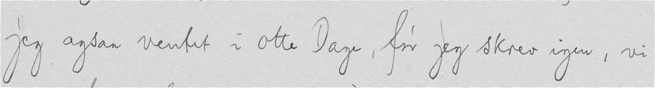jeg ogsaa ventet i otte Dage, før jeg skrev igen, vi
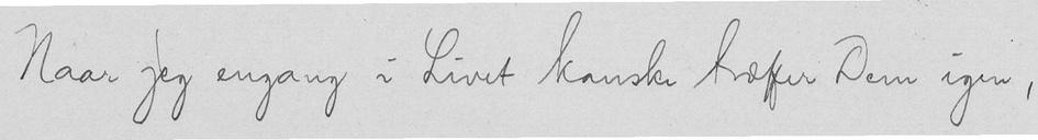Naar jeg engang i Livet kanske træffer Dem igen,
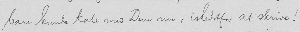bare kunde tale med Dem nu, istedetfor at skrive!
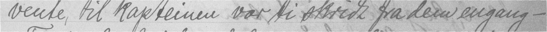vente, til kapteinen var ti skridt fra dem engang -
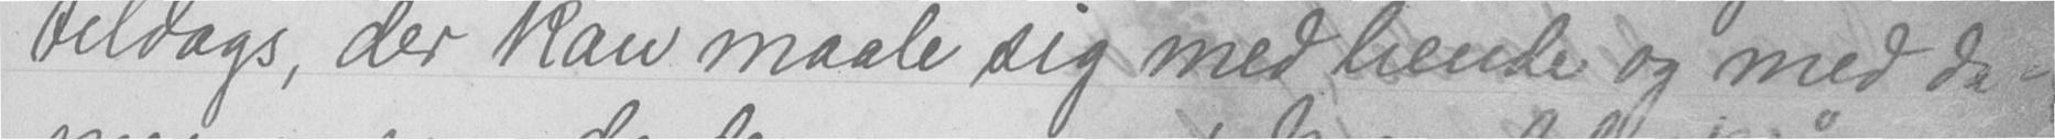tildags, der kan maale sig med hende og med da-
